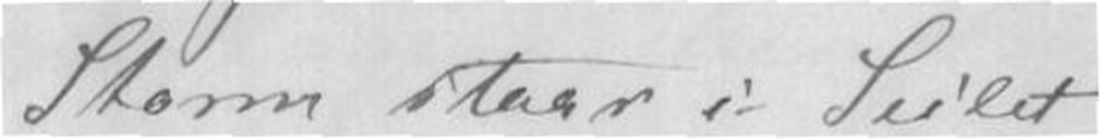Storm staar i Seilet,
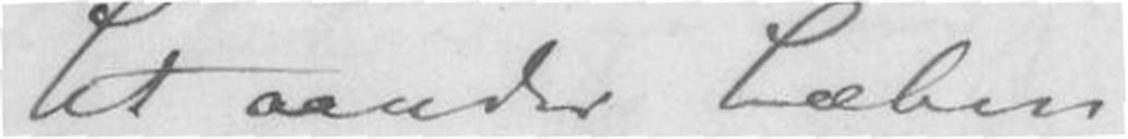Lit aander Læben
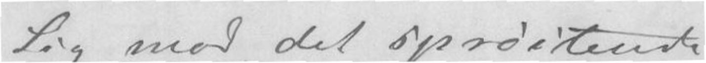Sig mod det sprøitende
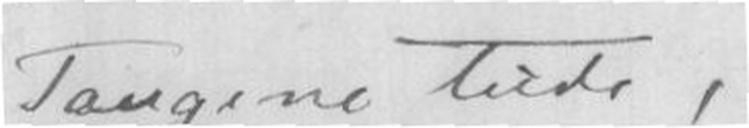Tangene tude,
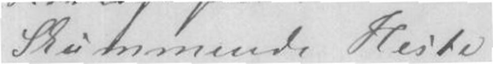Skummende Heste
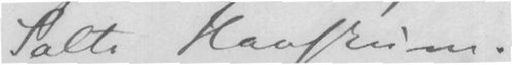Salte Havskum.
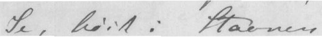Se, høit i Stavnen
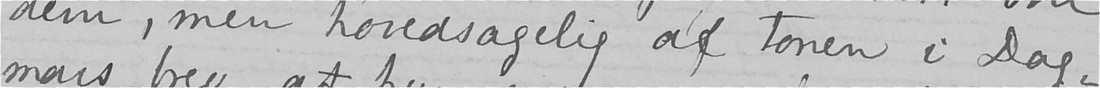dem, men hovedsagelig af tonen i Dag-
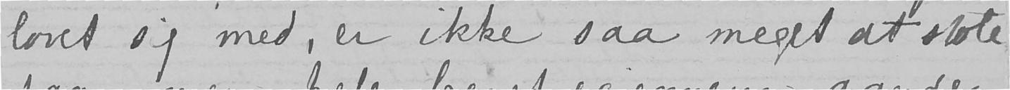lovet sig med, er ikke saa meget at stole
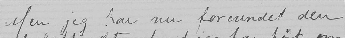Men jeg har nu forvundet den
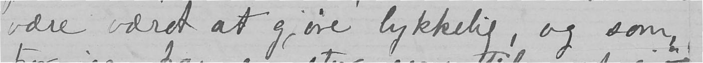være værdt at gjøre lykkelig, og som,
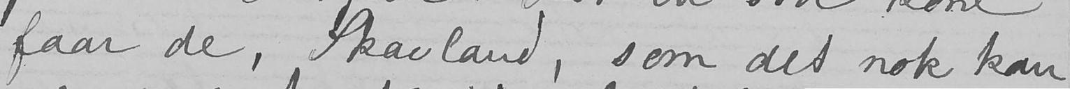faar de, Skavland, som det nok kan
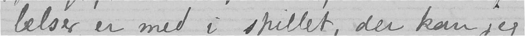lelser er med i spillet, der kan jeg
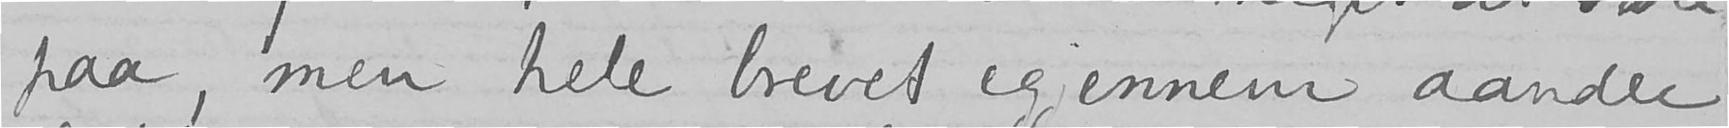paa, men hele brevet igjennem aander
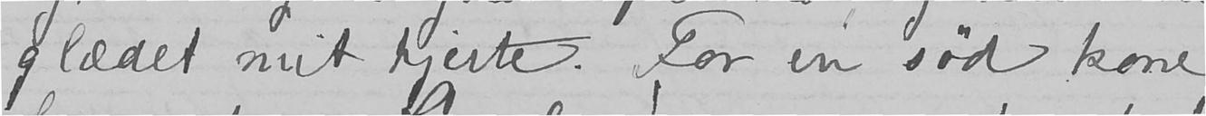glædet mit hjerte. For en sød kone
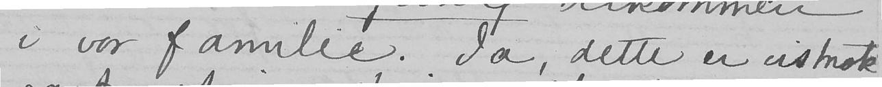i vor familie. Ja, dette er vistnok
